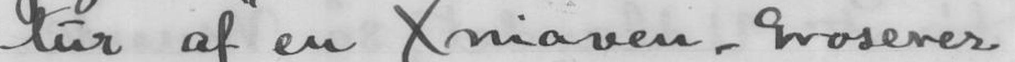tur af en Xniaven - Groserer
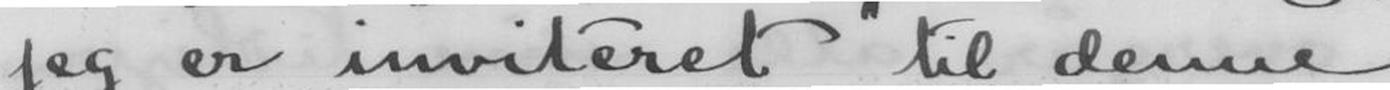jeg er inviteret til denne
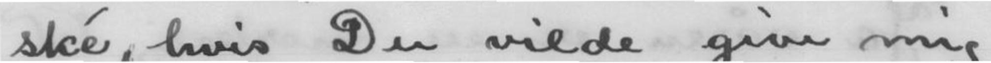ské, hvis Du vilde give mig
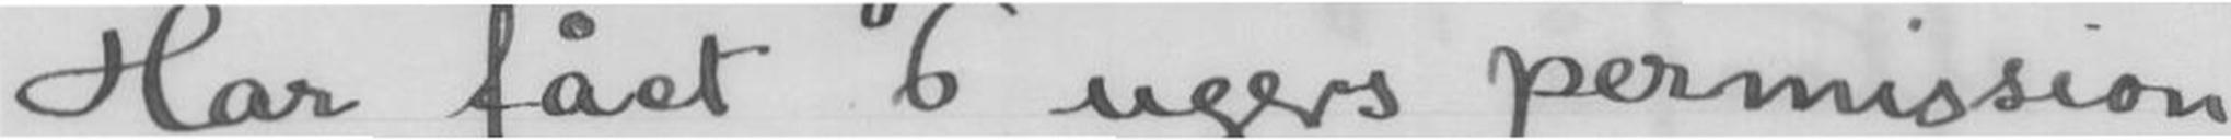Har fået 6 ugers permission
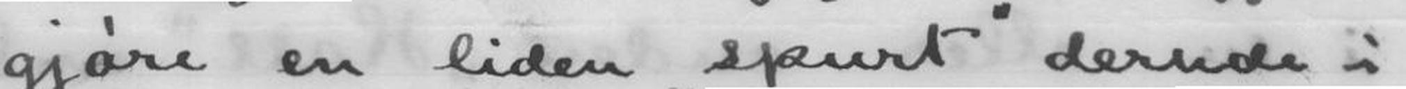gjøre en liden spurt derude i
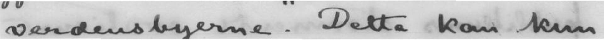verdensbyerne. Dette kan kun
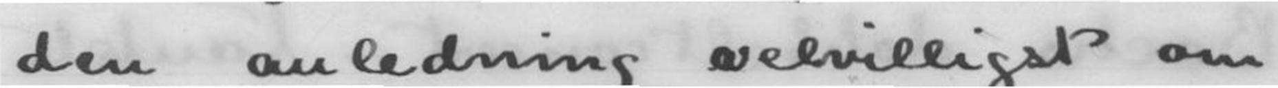den anledning velvilligst om
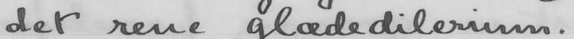det rene glædedilerium.
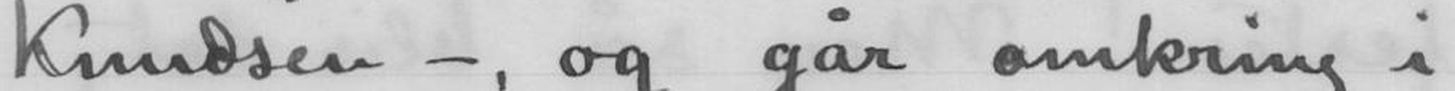Knudsen -, og går omkring i
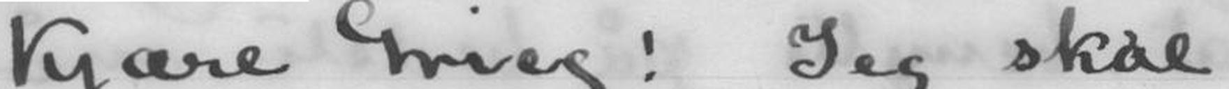Kjære Grieg!Jeg skal
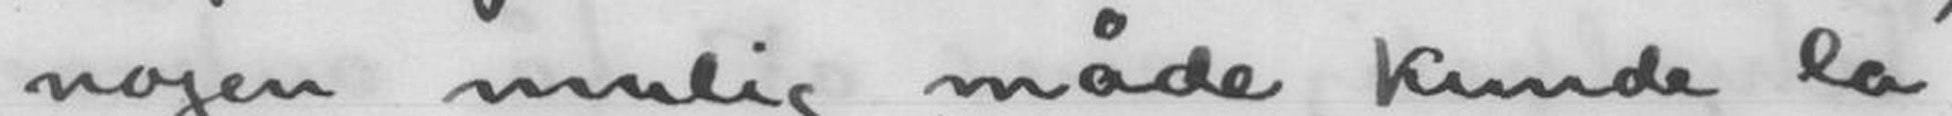nogen mulig måde kunde la'
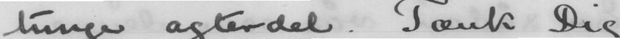tunge agterdel. Tænk Dig
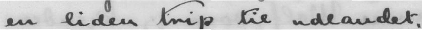en liden trip til udlandet, -
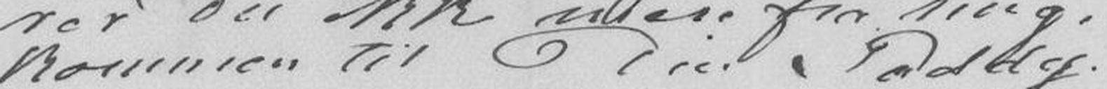kommen til Din Paddy.
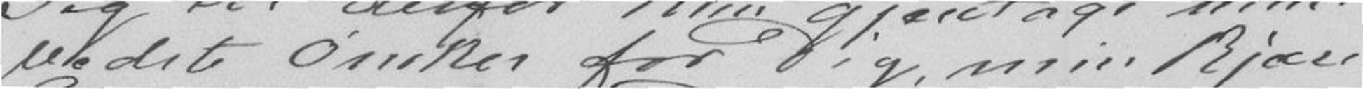bedste Ønsker for Dig, min kjære
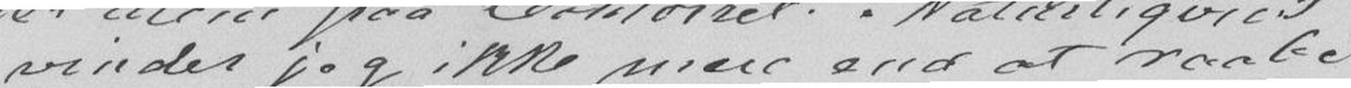vinder jeg ikke mere end at raabe
